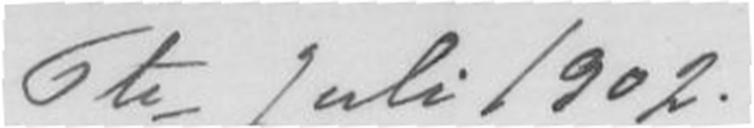6te Juli 1902.
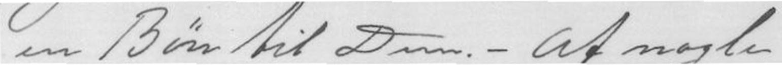en Bøn til Dem. - Af nogle
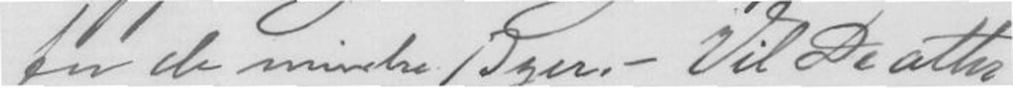for de mindre Byer. - Vil De atter
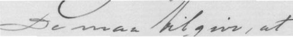De maa tilgive, at
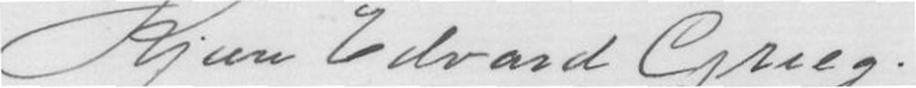Kjære Edvard Grieg.
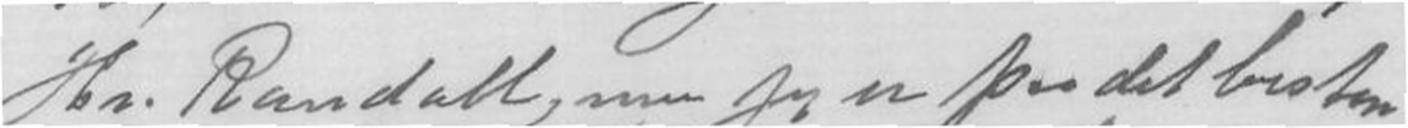Hr. Randall, men jeg er paa det bestem-
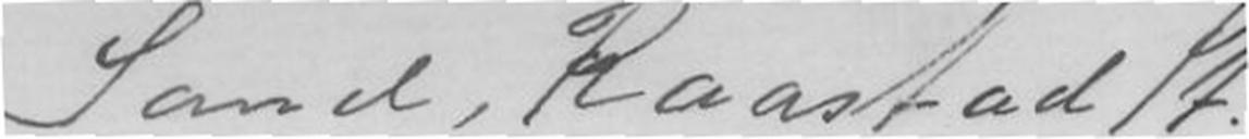Sand, Raastad St.
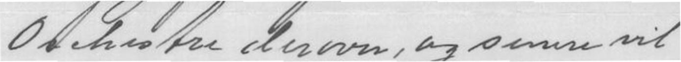Orchestre derover, og senere vil
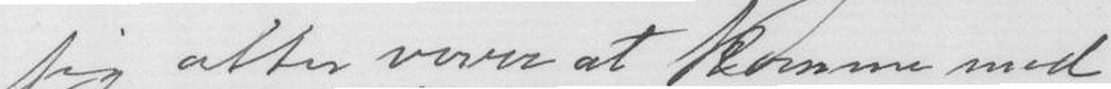jeg atter vover at komme med
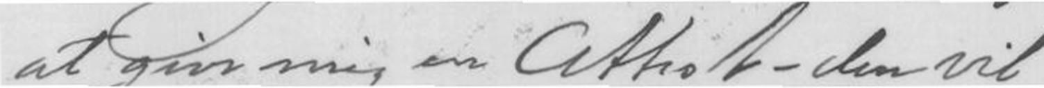at give mig en Attest der vil
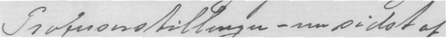Programstillingen - nu sidst af
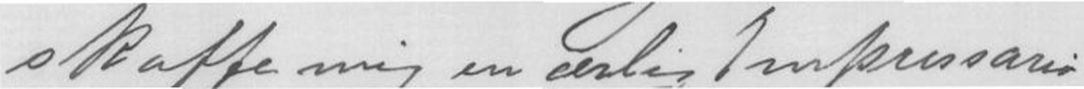skaffe mig en ærlig Impressario
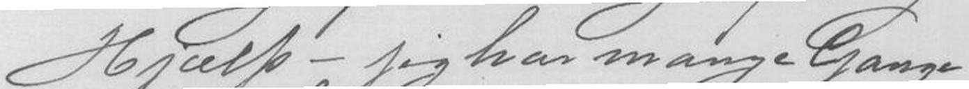Hjælp - jeg har mange Gange
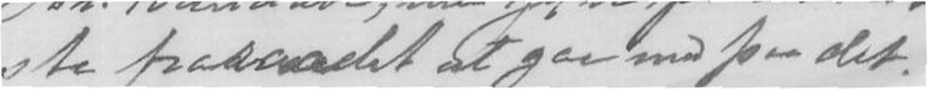ste fraraadet at gaa med paa det.
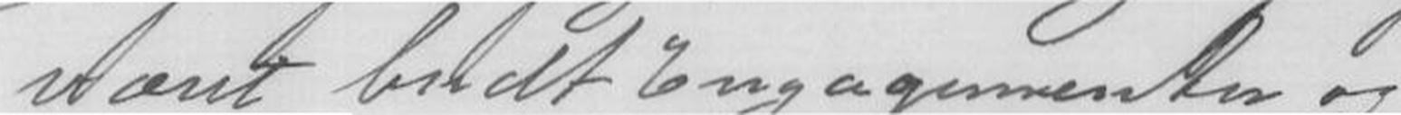været budt Engagementer og
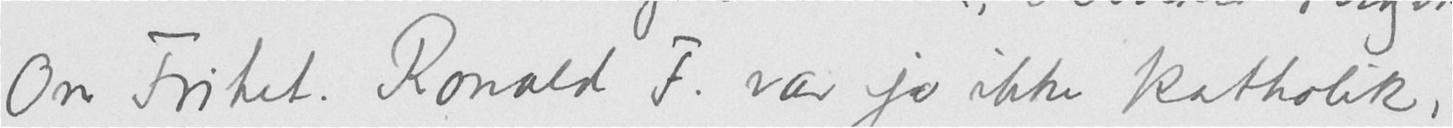Om Frihet. Ronald F. var jo ikke katholik,
